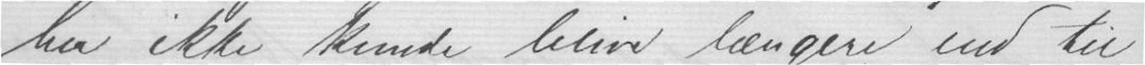her ikke kunde blive længer end til
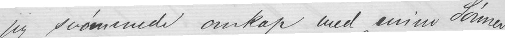jeg svømmede omkap med mine Sønner,
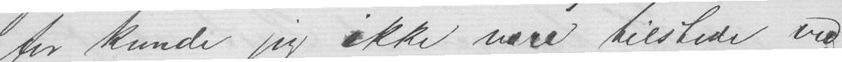for kunde jeg ikke være tilstede ved
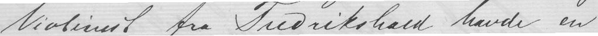Violinist fra Fredrikshald havde en
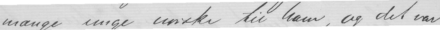mange unge norske til ham, og det var
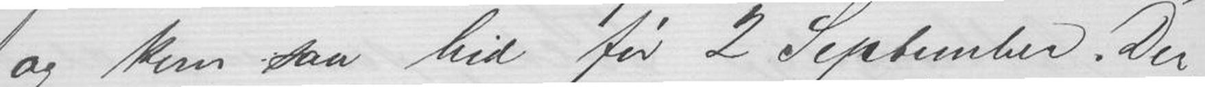og kom saa hid før 2 September. Der-
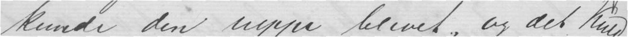kunde den neppe blivet, og det Kuld
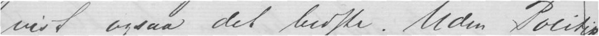vist ogsaa det bedste. Uden Politik
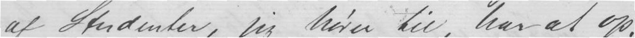af Studenter, jeg hører til, har at op-
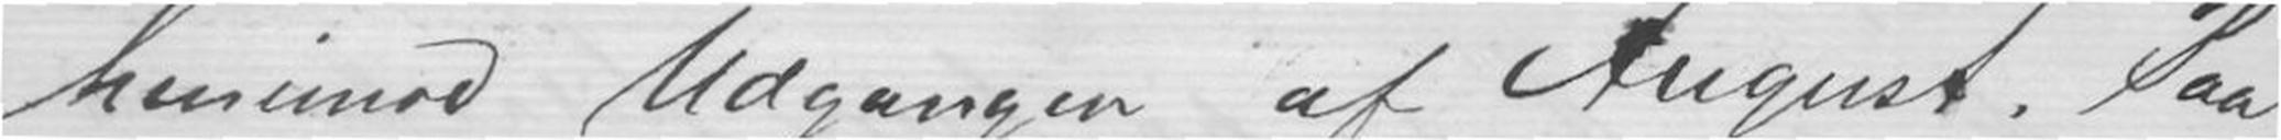henimod Udgangen af August. Paa
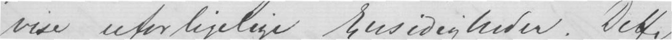vise uforligelige Ensidigheder. Dette
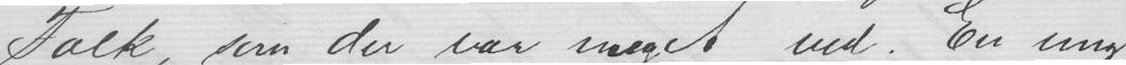Folk som der var meget ved. En ung
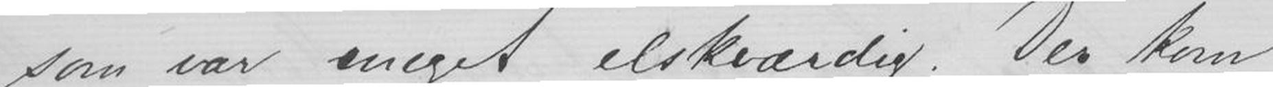som var meget elskværdig. Der kom
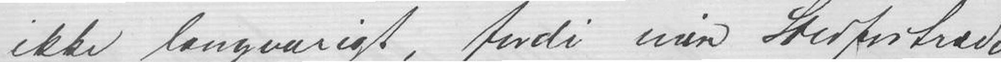ikke langvarigt, fordi min Stedfortræder
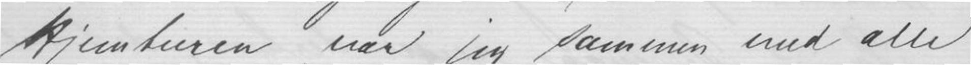Hjemturen var jeg sammen med alle
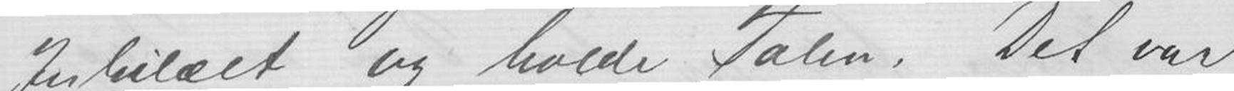Jubilæet og holde Talen. Det var
